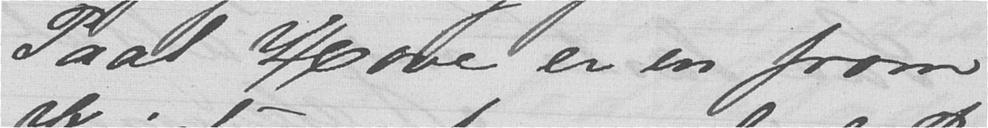Paal Hove er en from
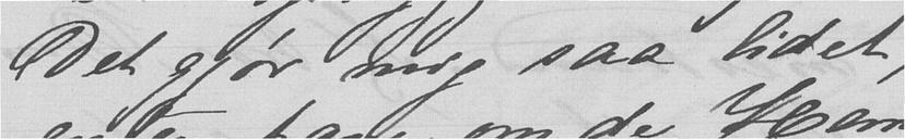Det gjør mig saa lidet,
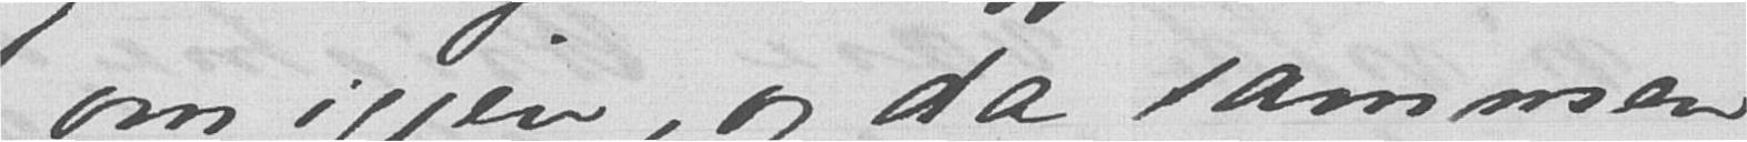om igjen, og da sammen
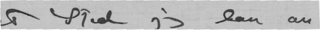et Sted jeg kan an-
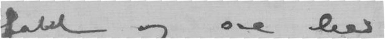fuld og saa har
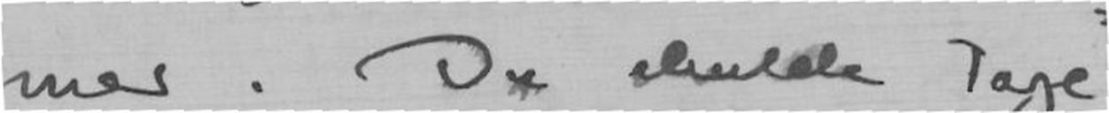mer. Du skulde Tage
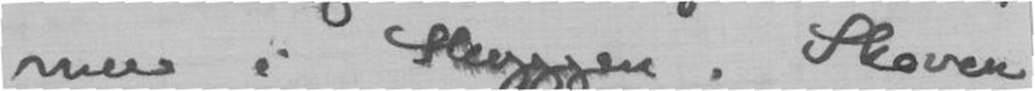mer i Skyggen. Skoven
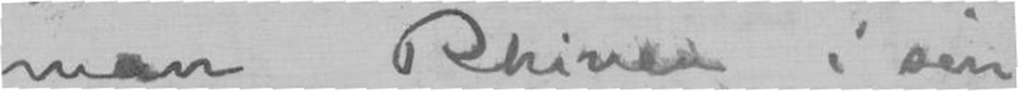man Rhinen i sin
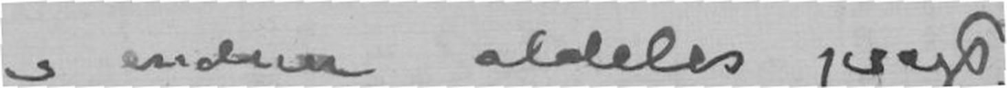er endnu aldeles pragt-
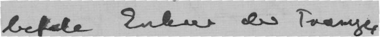befale Enhver der Trænger
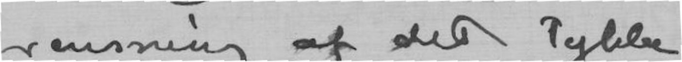vanning af det Tykke
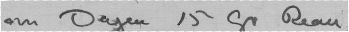om Dagen 15 Gr Reau-
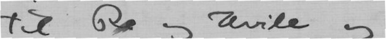til Ro og Hvile og
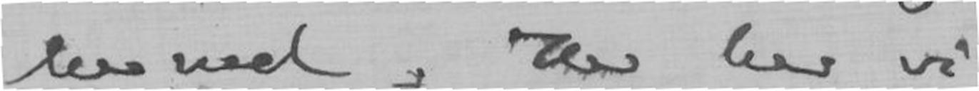her ned - Her har vi
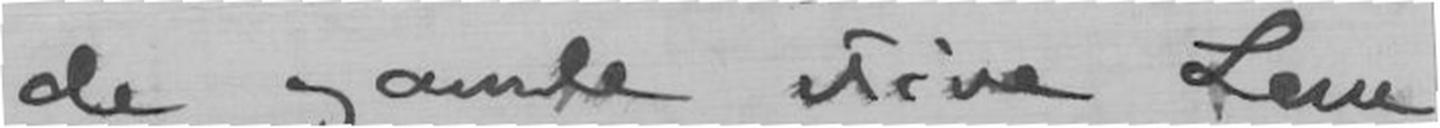de gamle stive Lem-
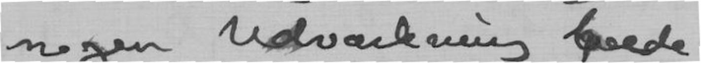nogen Udvaskning baade
